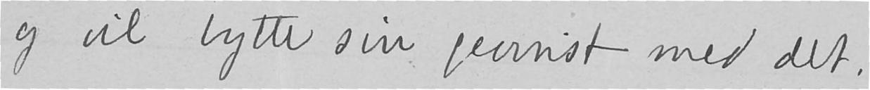og vil bytte sin gevinst med det.
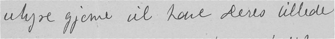uhyre gjerne vil have Deres billede,
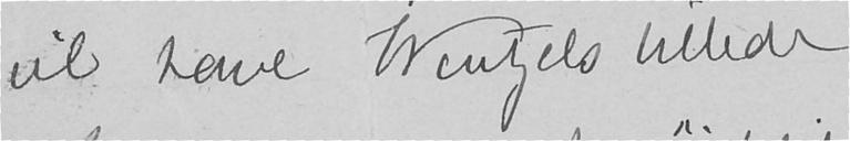vil have Wentzels billede.
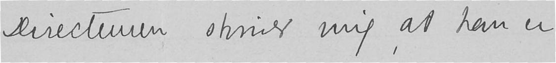Directeuren skriver mig, at han er
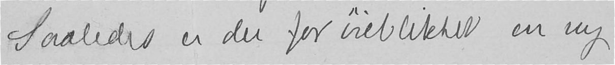Saaledes er der for øieblikket en ny
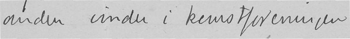anden vinder i kunstforeningen
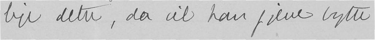lige dette, da vil han gjerne bytte
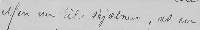Men nu til skjæbnen, at en
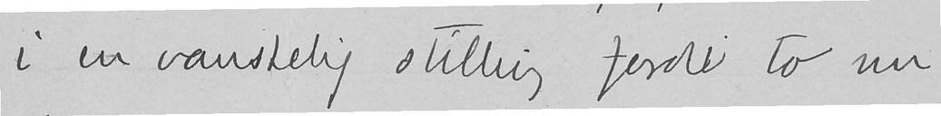i en vanskelig stilling fordi to nu
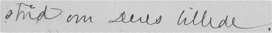strid om Deres billede.
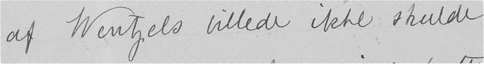af Wentzels billede ikke skulde
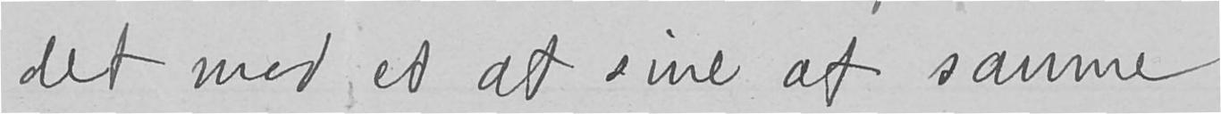det med et af sine af samme
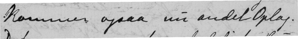kommer ogsaa nu andet Oplag.
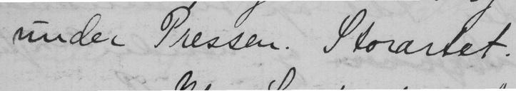under Pressen. Storartet.
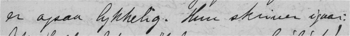er ogsaa lykkelig. Hun skriver igaar:
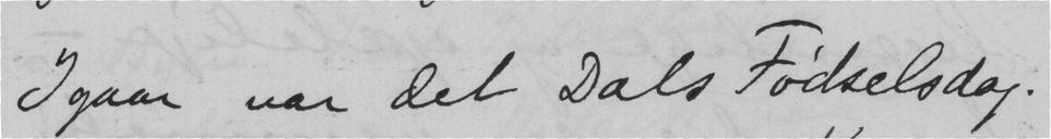Igaar var det Dals Fødselsdag.
